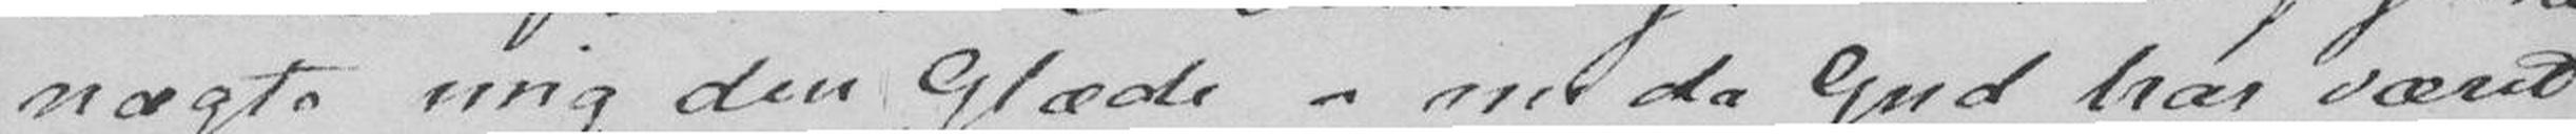nægte mig den Glæde, nu da Gud har været
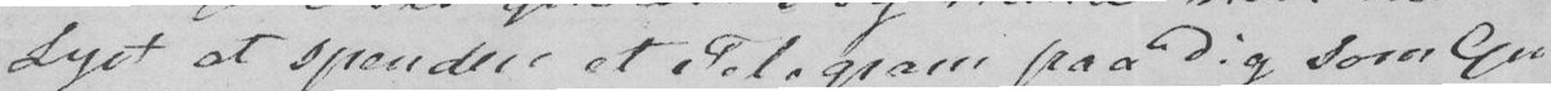Lyst at spendere et Telegram paa Dig som Be-
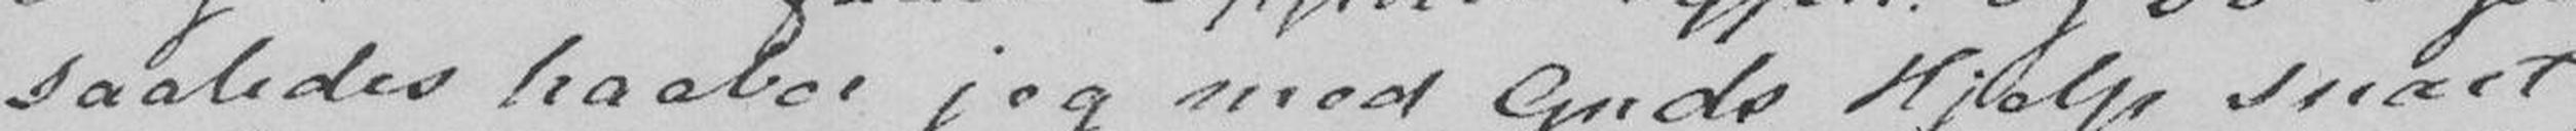saaledes haaber jeg med Guds Hjelp snart
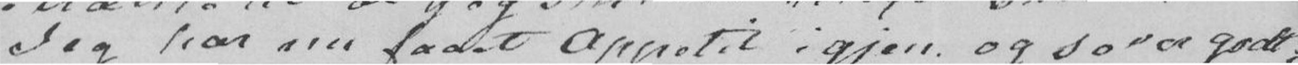Jeg har faaet Appetit igjen og sove godt;
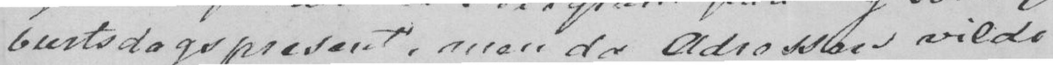bursdagspresent, men da Adressen vilde
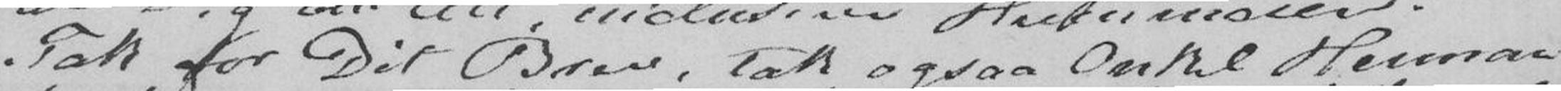Tak for Dit Brev, Tak ogsaa Onkel Hermen
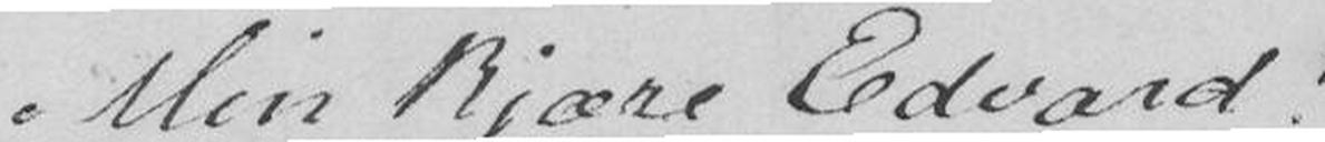Min kjære Edvard!
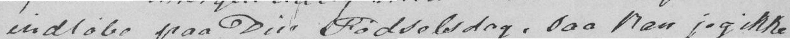indløbe paa Din Fødselsdag, saa kan jeg ikke
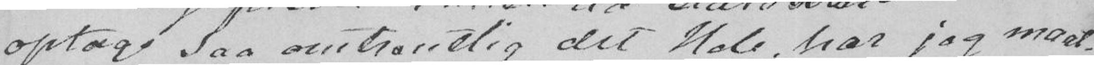optage saa omtrentlig det Hele, har jeg maat-
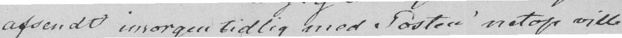afsendt imorgen tidlig med Posten netop ville
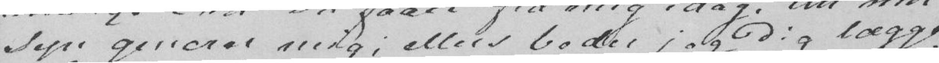Syn generer mig; ellers beder jeg Dig lægge
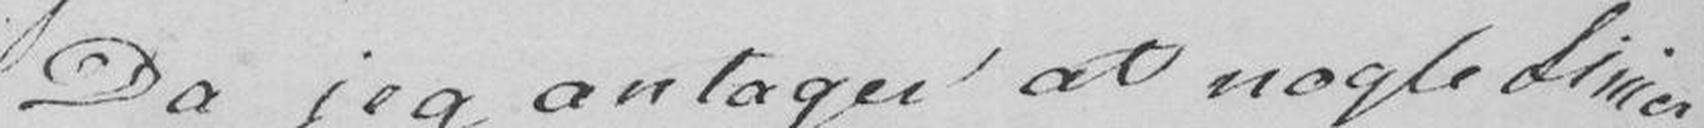Da jeg antager at nogle Linier
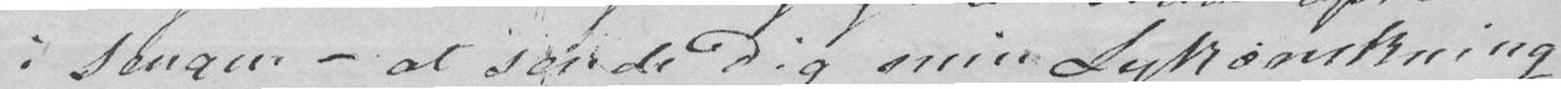i Sengen - at sende Dig min Lykønskning
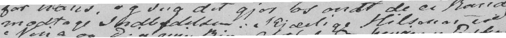modtage Indbydelsen: Kjærlige Hilsener til
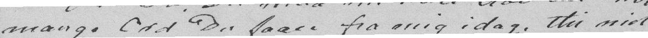mange Ord Du faaer fra mig idag, thi mit
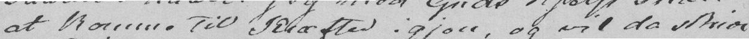at komme til Kræfter igjen, og vil da skrive
